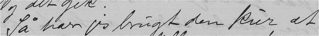Så har jeg brugt den kur at
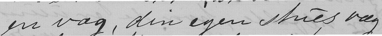en væg, din egen stues væg
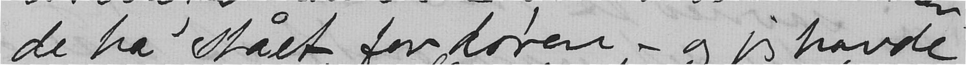de ha stået for døren - og jeg havde
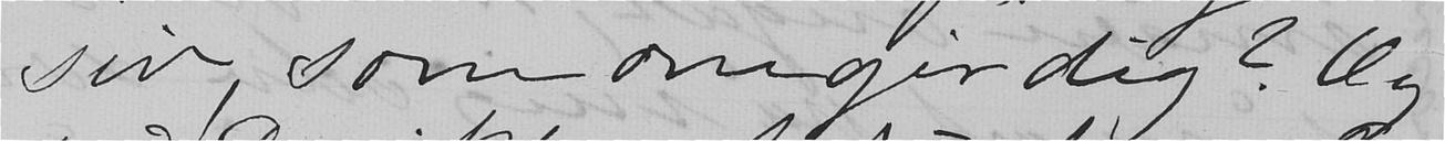siv som omgir dig? Og
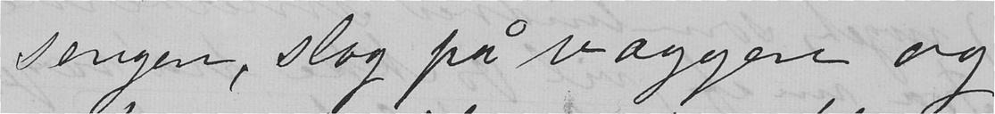sengen, slog på væggen og
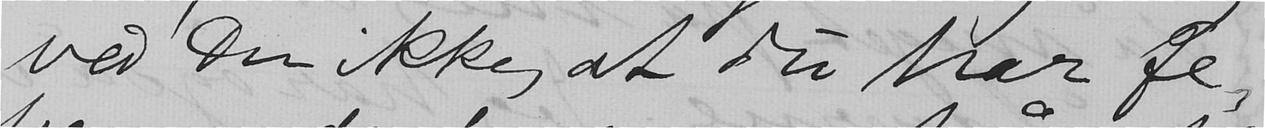ved Du ikke at du har fe-
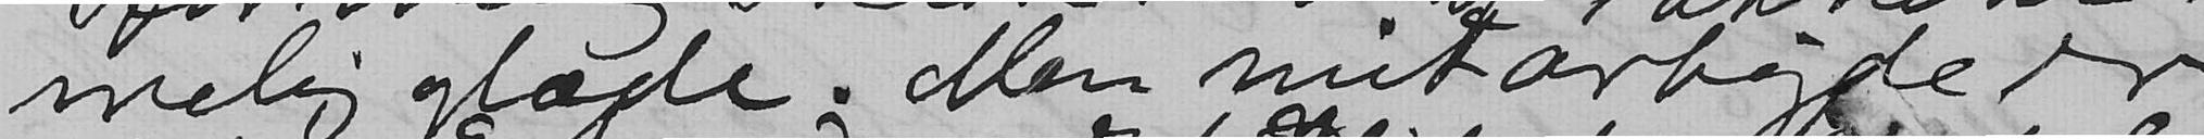melig glæde. Men mit arbejde er
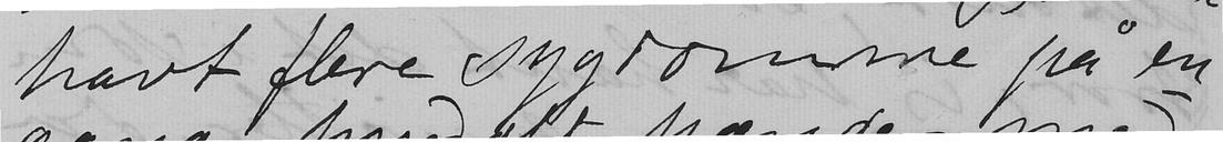havt flere sygdomme på en
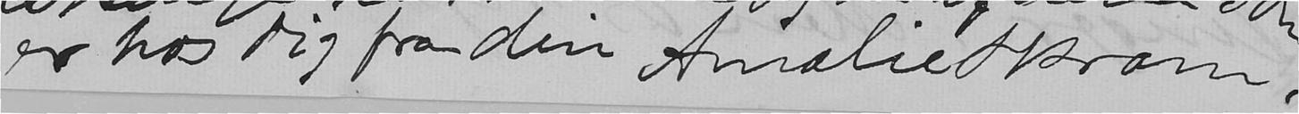er hos dig fra din Amalie Skram.
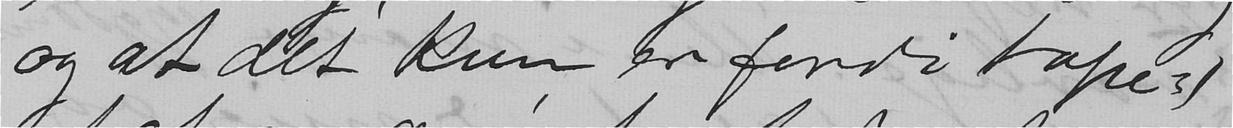og at det kun er fordi tape-
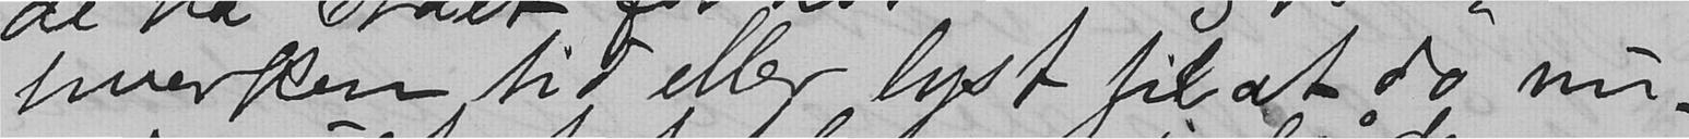hverken tid eller lyst til at dø nu,
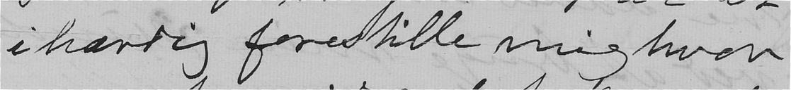ihærdig forestille mig hvor
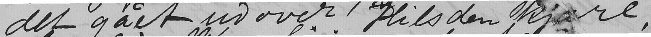det gået udover! Hils den kjære,
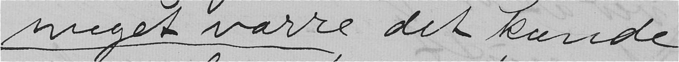meget værre det kunde
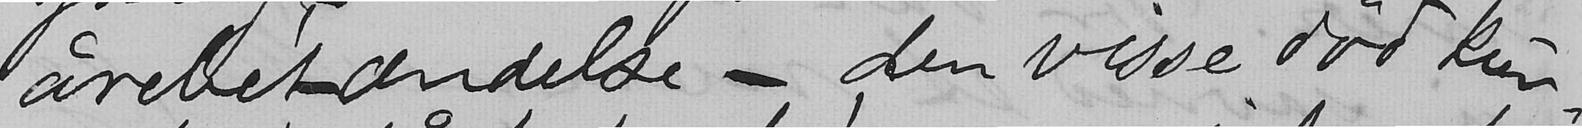årebetændelse - den visse død kun-
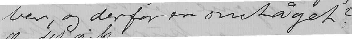ber, og derfor er omtåget?
